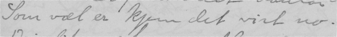Som væl er kjem det vist no.
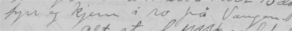fyrr eg kjem i ro frå Vangen.
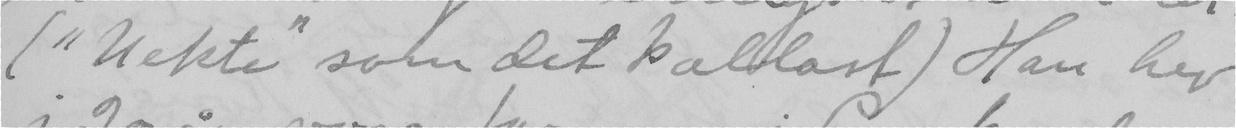("Uekte" som det kallast) Han hev
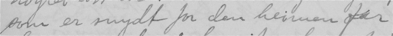som er snydt for den heimen far
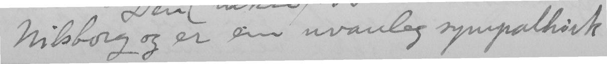Nilsborg og er ein uvanleg sympatisk
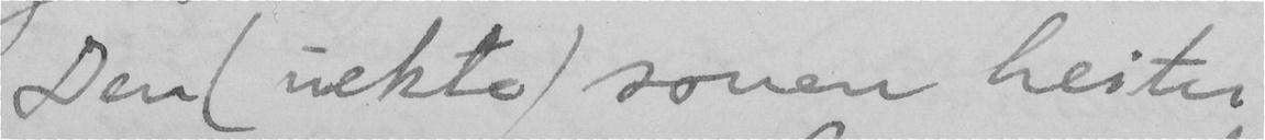Den (uekte) sonen heitar
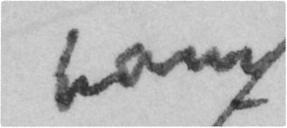ham
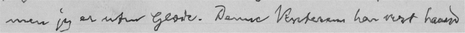men jeg er uten Glæde. Denne Vinterenn har vert haard
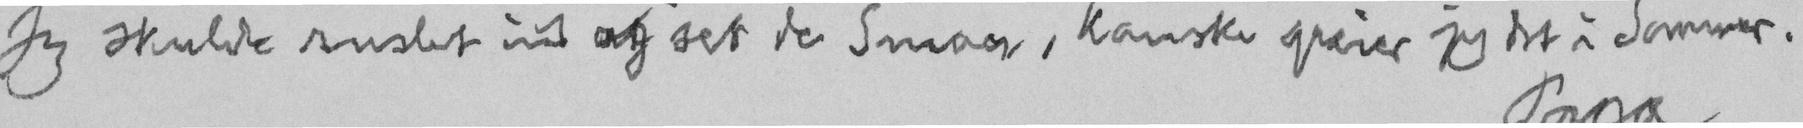Jeg skulde ruslet ind og set de Smaa, kanske greier jeg det i Sommer.
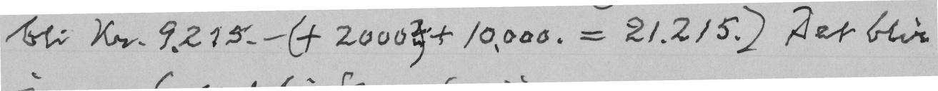bli Kr. 9.215. - (+ 2000 2). + 10.000. = 21.215.) Det blir
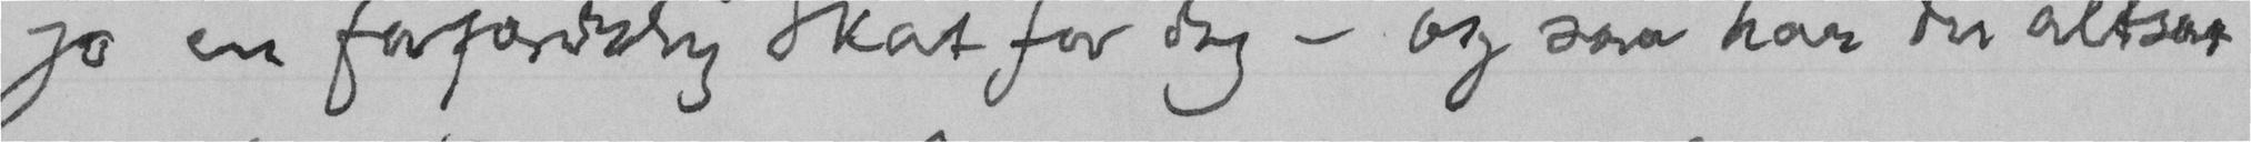jo en forfærdelig Skat for dig - og saa har du altsaa
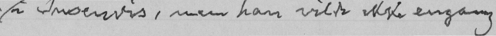i Tusenvis, men han vilde ikke engang
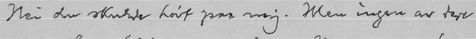Nei du skulde hørt paa mig. Men ingen av dere
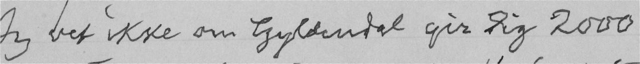Jeg vet ikke om Gyldendal gir dig 2000
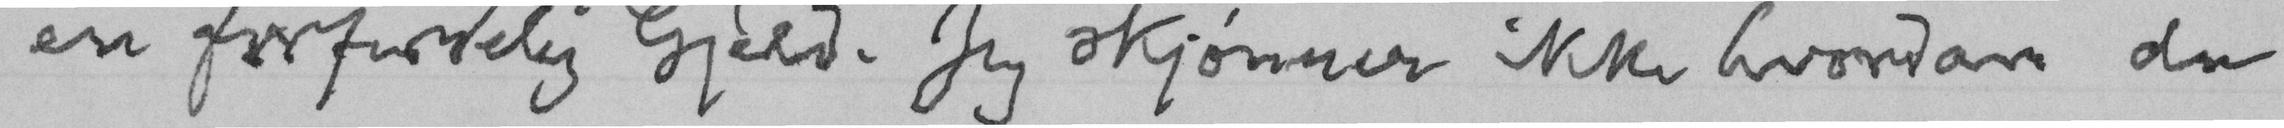en forferdelig Gjeld. Jeg skjønner ikke hvordan du
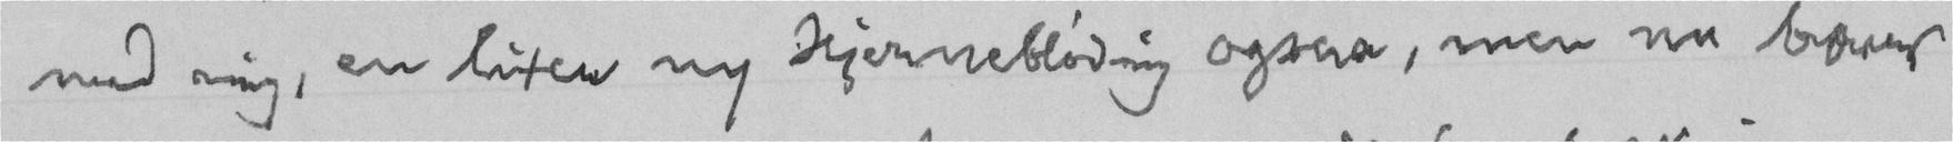med mig, en liten ny Hjerneblødning ogsaa, men nu bærer
